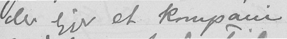der ligger et kompani
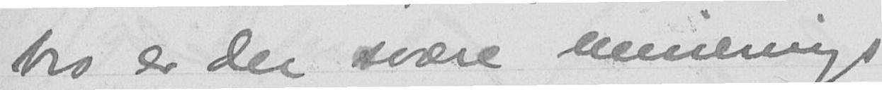bro er der svære menerings
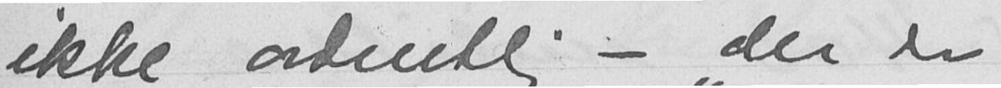ikke ordentlig - "der er
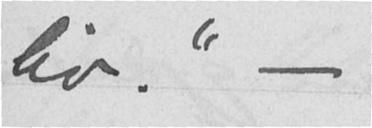liv." -
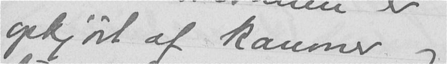opkjørt af kanoner og
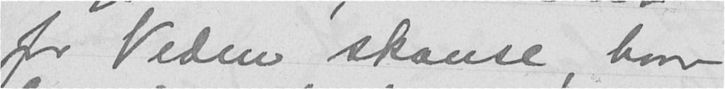for Veden skanse, hvor
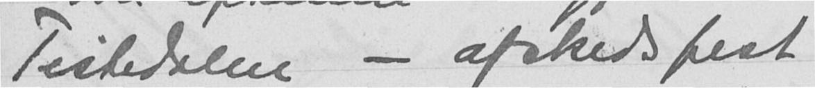Tistedalen - afskedsfest
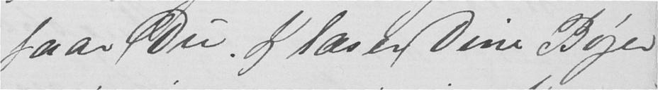faar Du. Jeg læser Dine Bøger
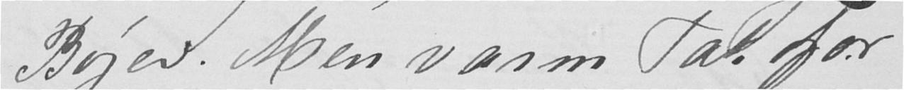Bøger. Men varm Tak for
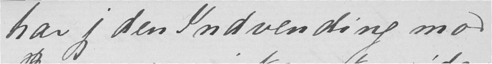har jeg den Indvending mod
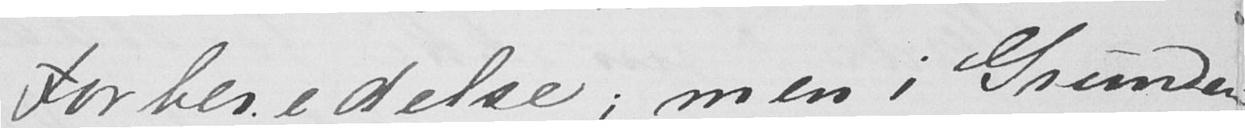Forberedelse; men i Grunden
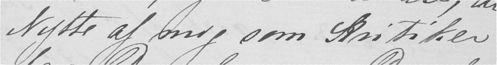Nytte af mig som Kritiker
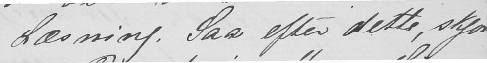Læsning. Saa efter dette, skjøn-
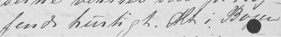fende hurtigt. Alt i Bogen
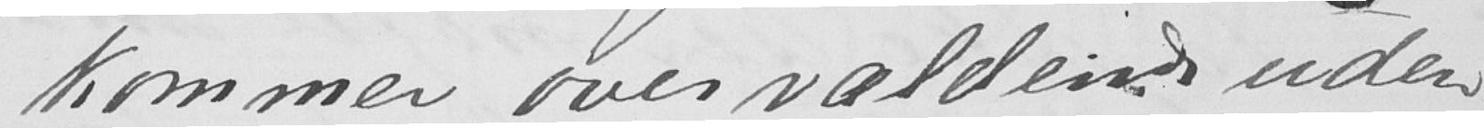kommer overvældende uden
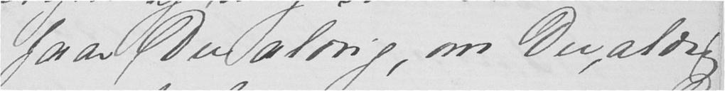faar Du aldrig, om Du, aldrig
